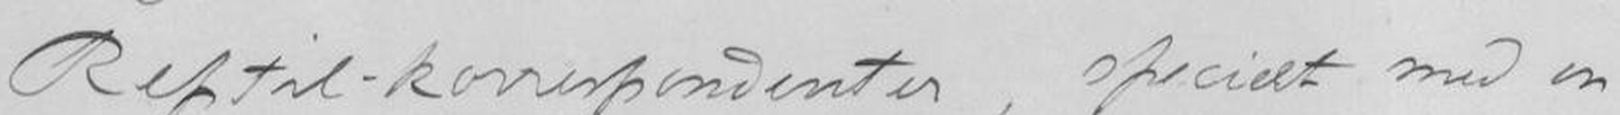Reptil-korrespondenter, specielt med en
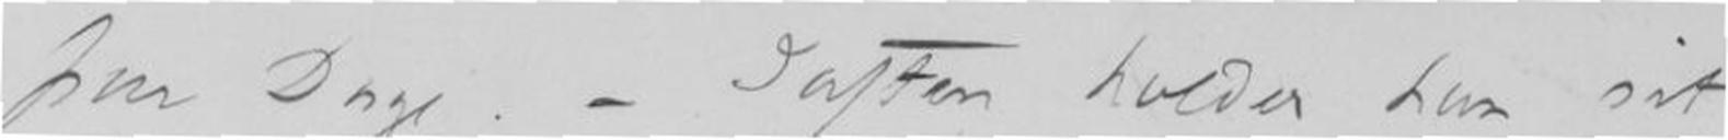par Dage. - Iaften holder han sit
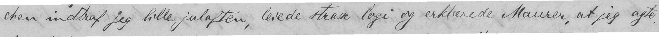chen indtraf jeg lille julaften, leiede strax logi og erklærede Maurer, at jeg agte-
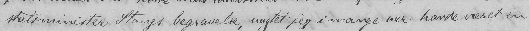statsminister Stangs begravelse, uagtet jeg i mange aar havde været en
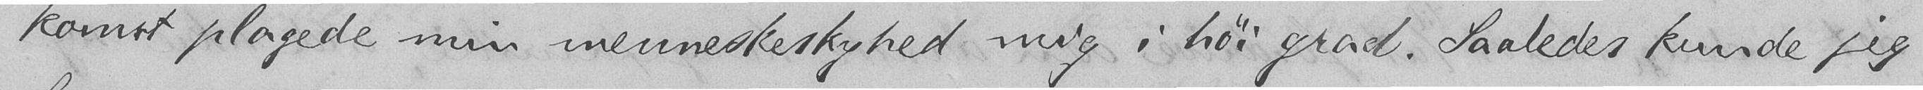komst plagede min menneskeskyhed mig i høi grad. Saaledes kunde jeg
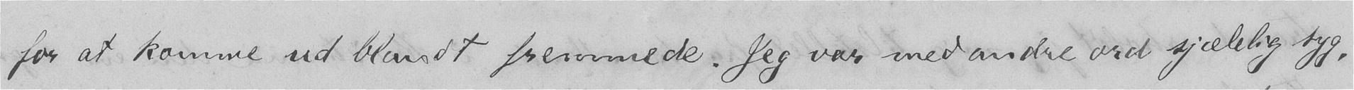for at komme ud blandt fremmede. Jeg var med andre ord sjælelig syg,
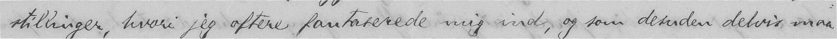stillinger, hvori jeg oftere fantaserede mig ind, og som desuden delvis maa
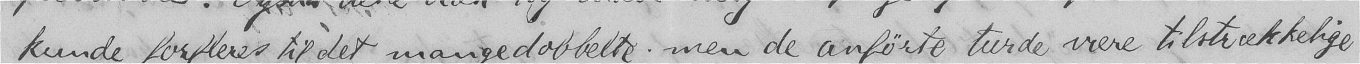kunde forfleres til det mangedobbelte; men de anførte turde være tilstrækkelige
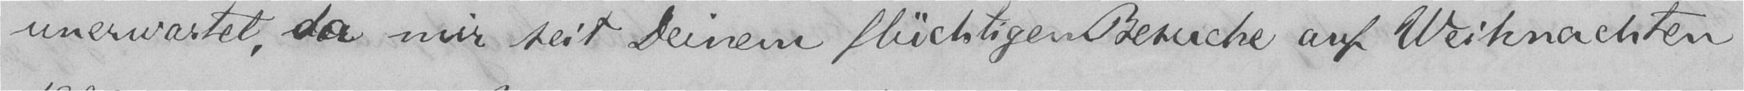unerwartet, da mir seit Deinem flüchtigen Besuche auf Weihnachten
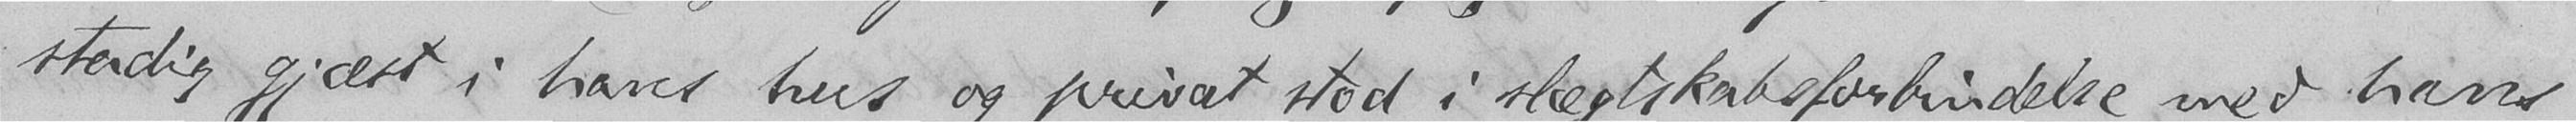stadig gjæst i hans hus og privat stod i slægtskabsforbindelse med hans
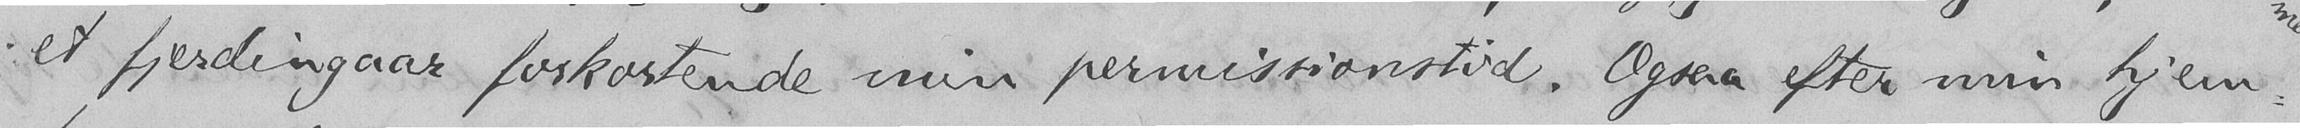et fjerdingaar forkortende min permissionstid. Ogsaa efter min hjem-
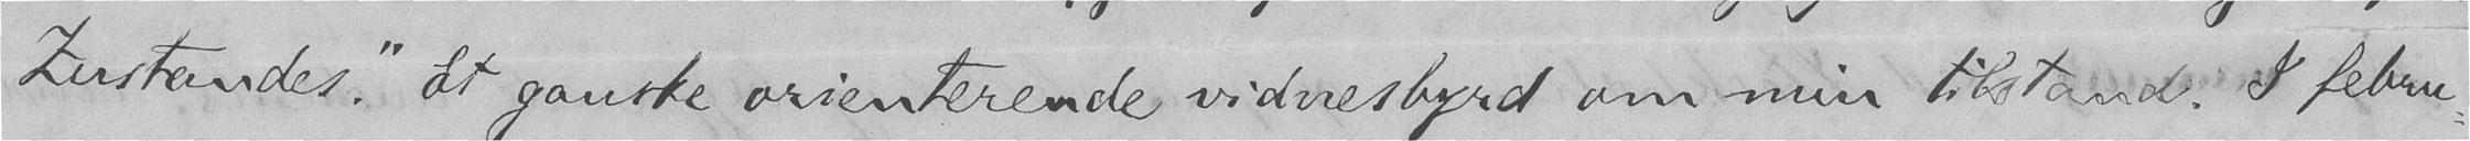Zustendes." Et ganske orienterende vidnesbyrd om min tilstand. I febru-
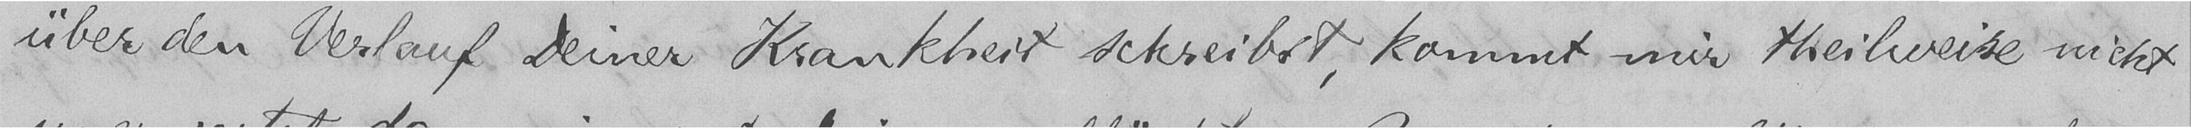uber den Verlauf Deiner Krankheit schreibst, kommt mir theilweise nicht
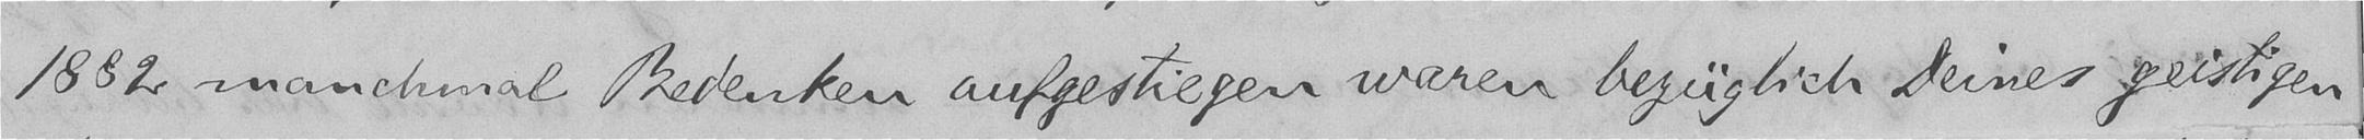1882 manchmal Bedenken aufgestiegen waren bezüglich Deines geistigen
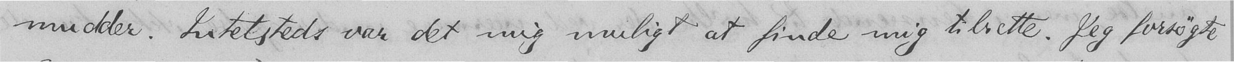mudder. Intetsteds var det mig muligt at finde mig tilrette. Jeg forsøgte
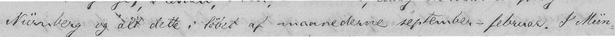Nürnberg og alt dette i løbet af maanederne september-februar. I Mün-
